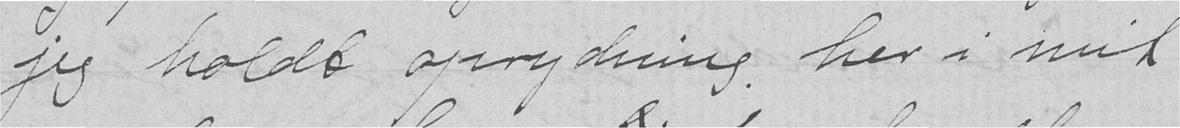jeg holdt oprydning her i mit
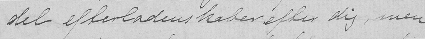del efterladenskaber efter dig, men
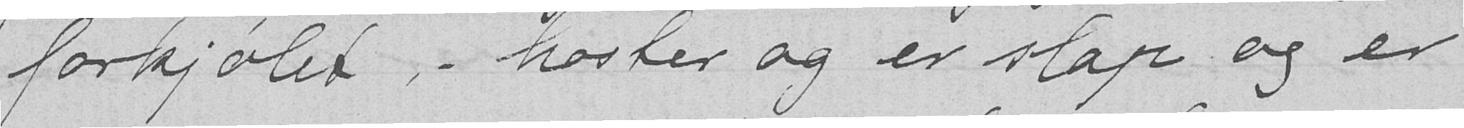forkjølet, - hoster og er slap og er
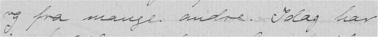og fra mange andre. Idag har
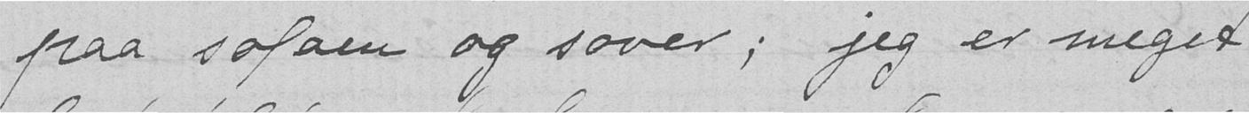paa sofaen og sover; jeg er meget
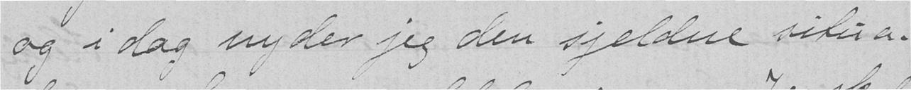og i dag nyder jeg den sjeldne situa-
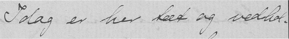Idag er her tæt og vedhol-
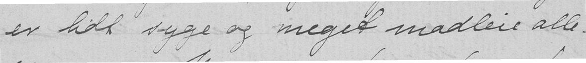er lidt syge og meget madleie alle-
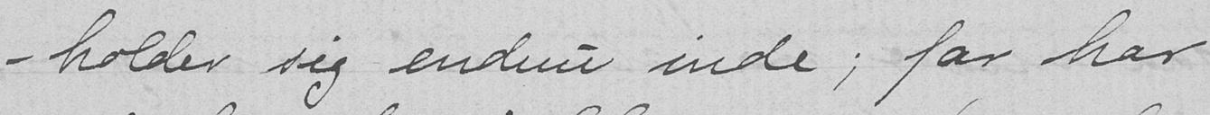- holder seg endnu inde, far har
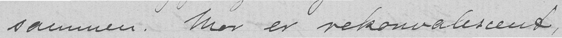sammen. Mor er rekonvalescent,
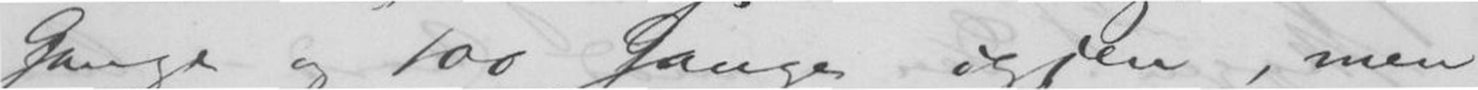Gange og 100 Gange igjen, men
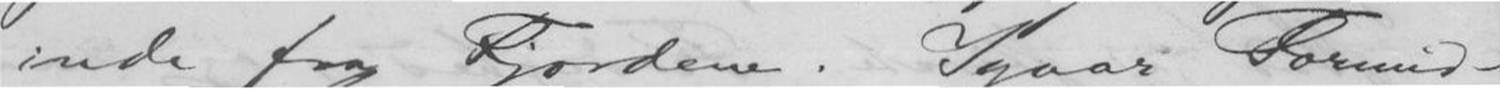inde fra Fjordene. Igaar Formid-
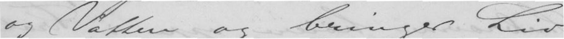og Vatten og bringer Liv
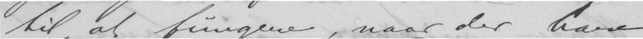til at fungere, naar der bare
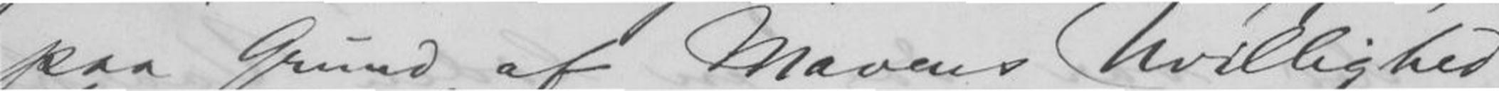paa Grund af Mavens Uvillighed
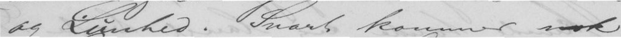og Lunhed. Snart kommer nok
